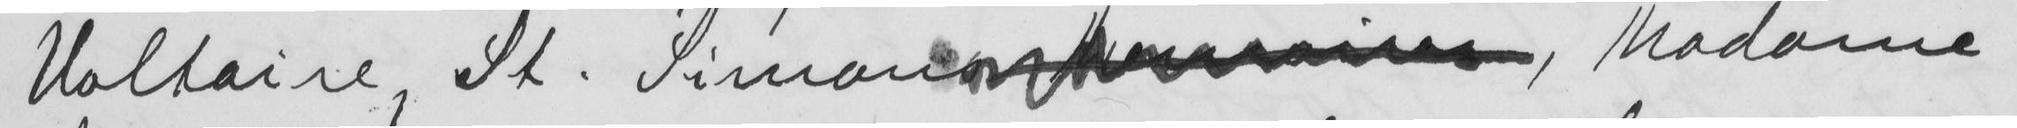Voltaire St. Simon, Madame
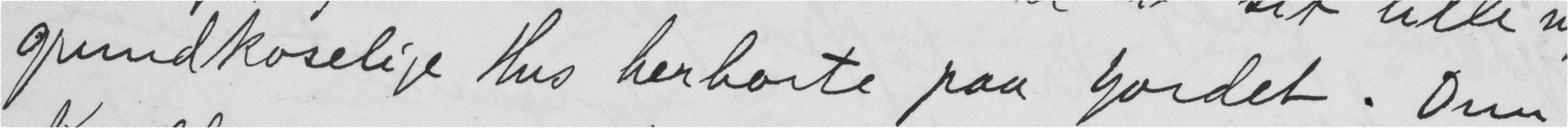grundkoselige Hus herborte paa Jordet. Den
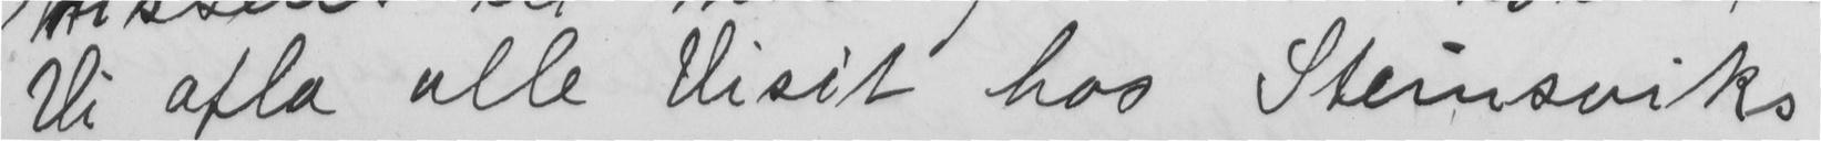Vi afla alle Visit hos Steisviks
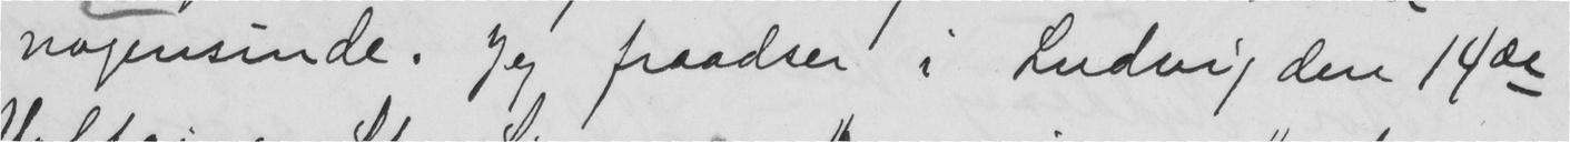nogensinde. Jeg fraadser i Ludvig den 14de
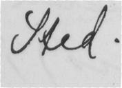Sted.
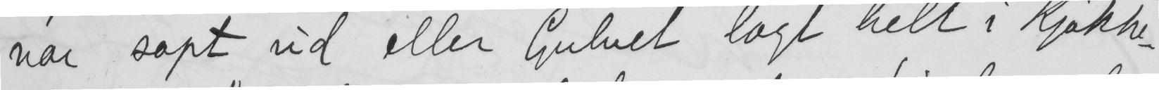var sopt ud eller Gulvet lagt helt i Kjøkke-
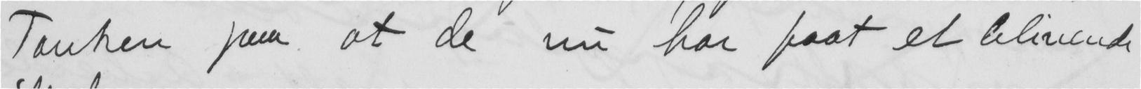Tanken paa at de nu har faat et blivende
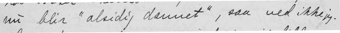nu blir "alsidig dannet", saa ved ikke jeg.
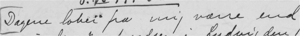Dagene løber fra mig værre end
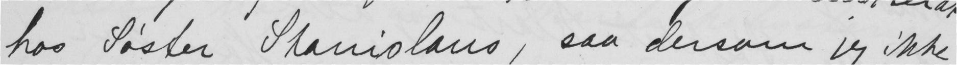hos Søster Stanislaus, saa dersom jeg ikke
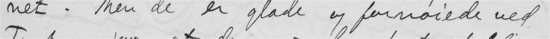net. Men de er glade og fornøiede ved
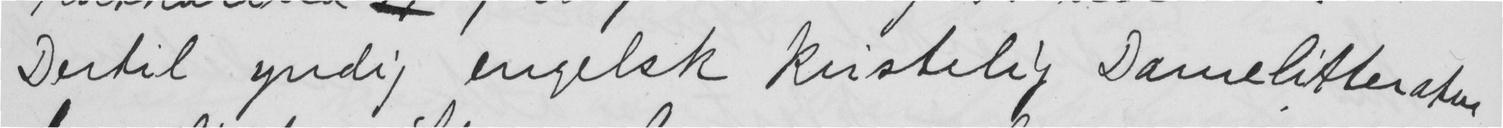Dertil yndig engelsk Kristelig Damelitteratur
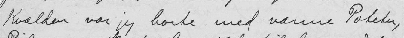Kvælden var jeg borte med varme Poteter,
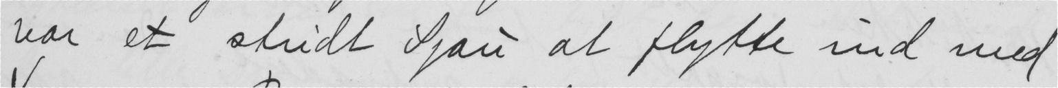var et studt Sjau at flytte ind med
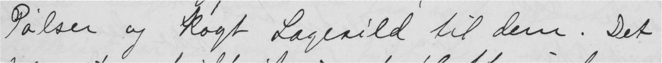Pølser og Rogt Lagesild til dem. Det
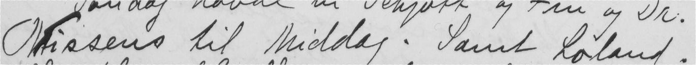Nissens til Middag. Samt Løland.
